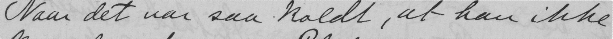Naar det var saa koldt, at han ikke
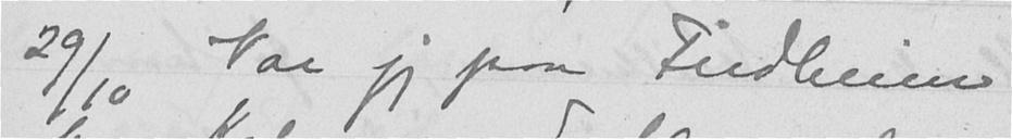29/10 Var jeg paa Fridheim
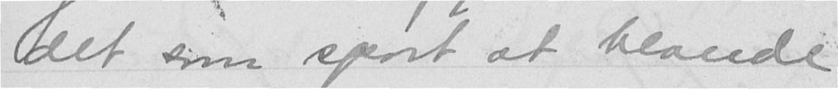det som sport at blande
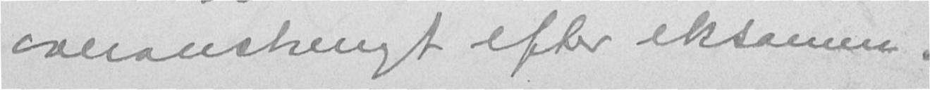overanstrengt efter eksamen.
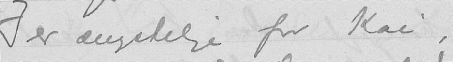er ængstelige for Kai,
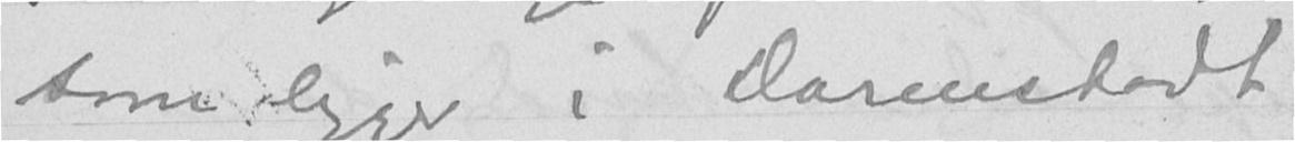som ligger i Darmstadt
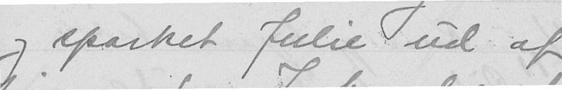og sparket Julie ud af
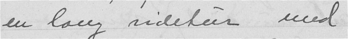en lang ridetur med
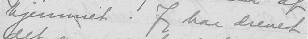hjemmet. Jeg har drevet
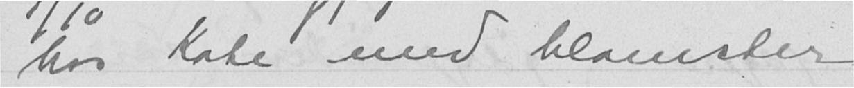hos Kate med blomster
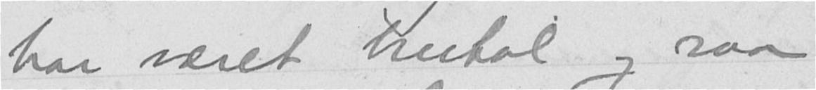har været brutal og raa
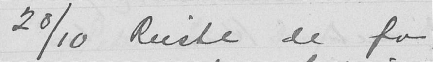28/10 Reiste de to
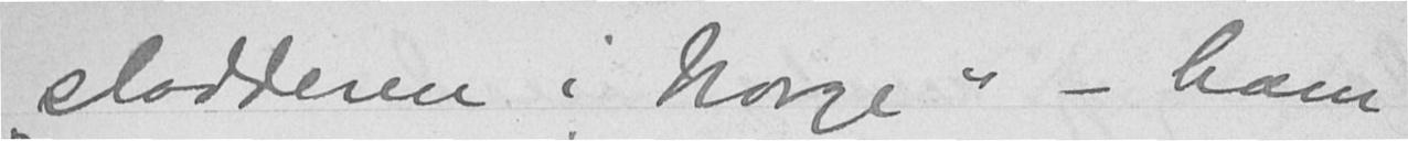"sladderen i Norge" - ham
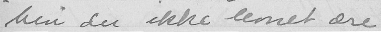blir der ikke levnet ære
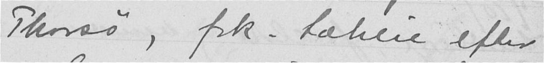Thorsø, frk. Sæhlie efter
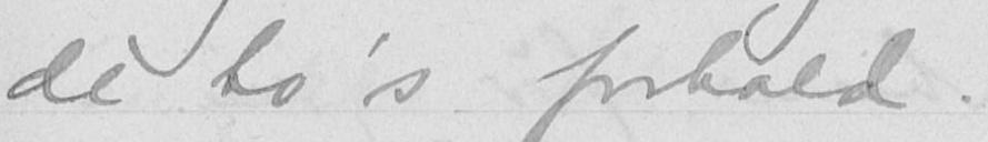de to's forhold.
